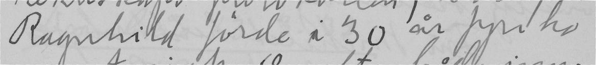Ragnhild förte i 30 år fyrr ho
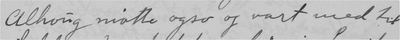Alhoug mötte også og vart med til
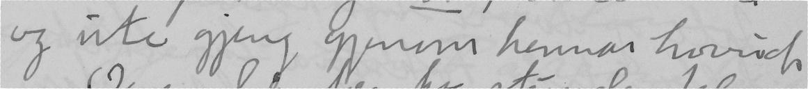og ute gjeng gjenem hennar hovud.
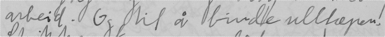arbeid. Og til å binde ulltæpen!
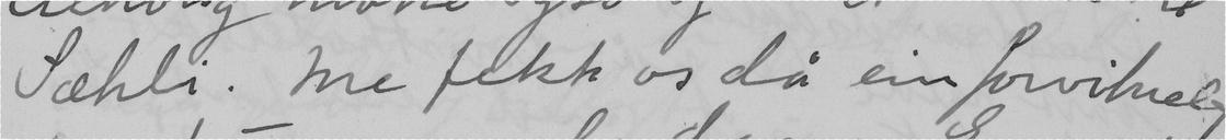Sæhli. Me fikk os då ein forvitneleg
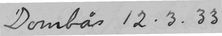Dombås 12.3.33
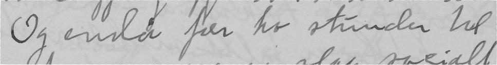Og enda får ho stunder til
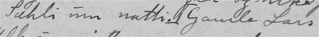Sæhli um natti. Gamle Lars
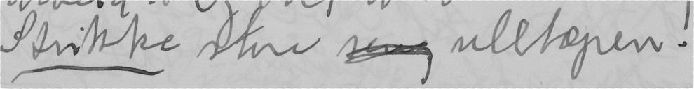Strikke store  ulltæpen!
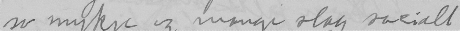so mykje og mange slag socialt
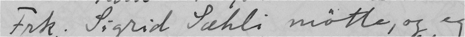Frk. Sigrid Sæhli møtte, og eg
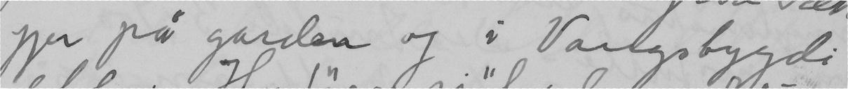gjer på garden og i Vangsbygdi
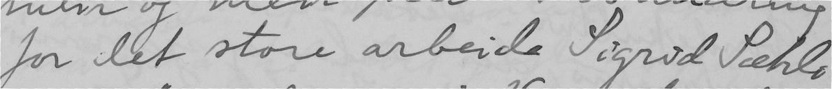for det store arbeide Sigrid Sæhli
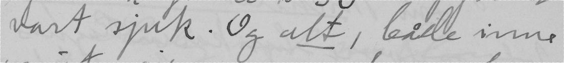vart sjuk. Og alt, både inne
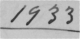1933
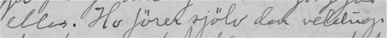ellers. Ho förer sjölv den veldugi
